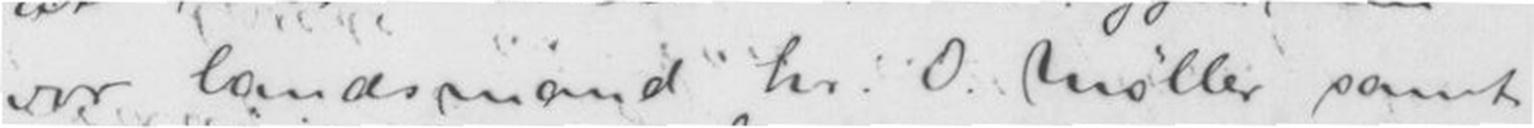vor landsmand hr. O. Møller samt
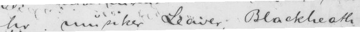hr. musiker Leaver, Blackheath,
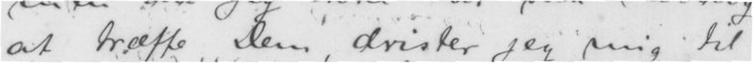at træffe Dem, drister jeg mig til
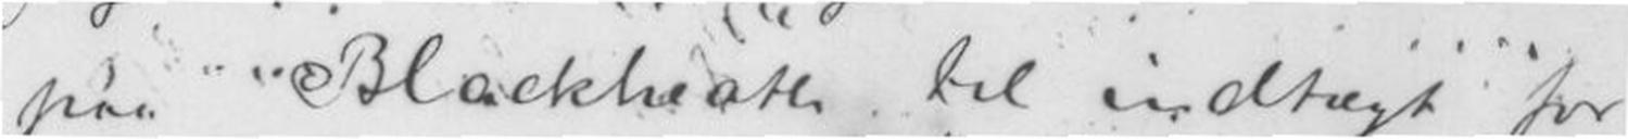paa Blackheath til indtækt for
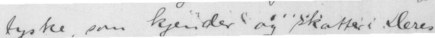tyske, som kjender og skatter Deres
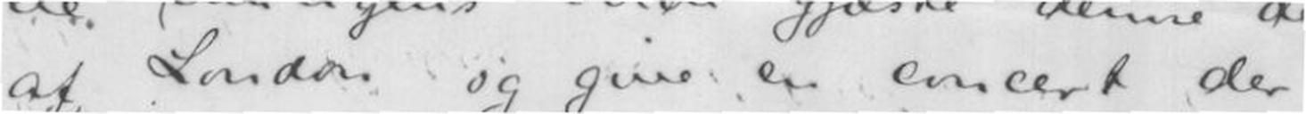af London og give en concert der
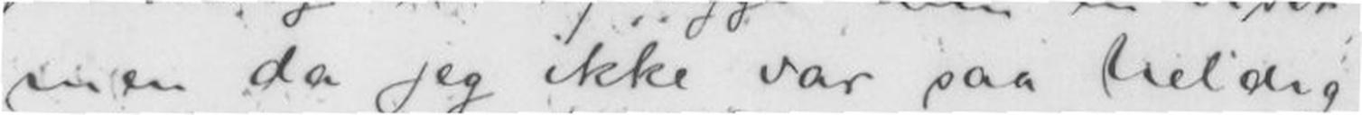men da jeg ikke var saa heldig
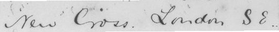New Cross London S E
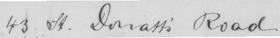43 St. Donatt's Road
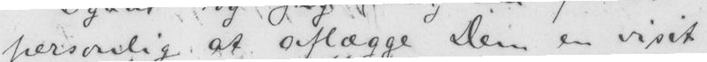personlig at aflægge Dem en visit
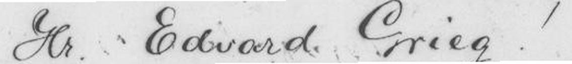Hr. Edvard Grieg!
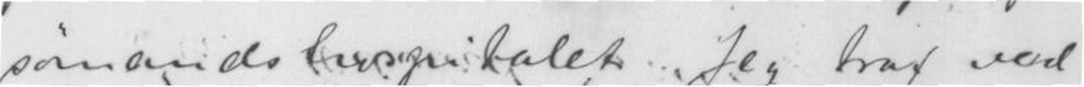sømandshospitalet. Jeg traf ved
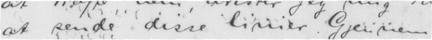at sende disse linier Gjennem
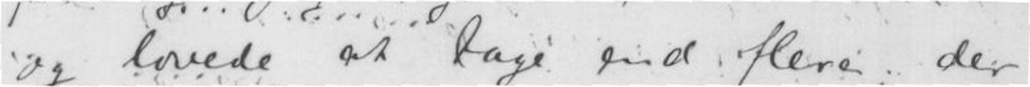og lovede at tage end flere, der
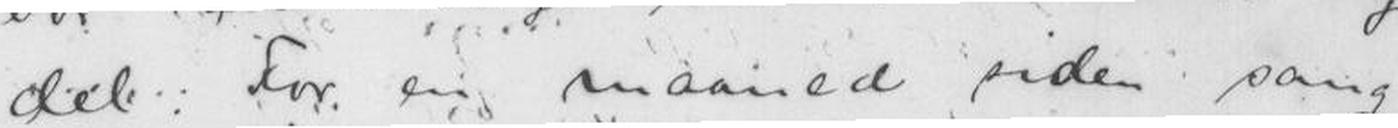del. For en maaned siden sang
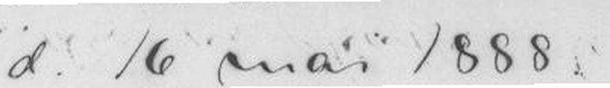d. 16 mai 1888
